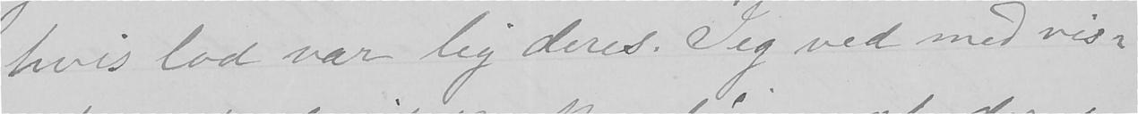hvis lod var lig deres. Jeg ved med vis-
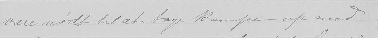være nødt til at tage kampen op mod.
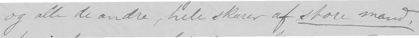og alle de andre, hele skarer af store mænd,
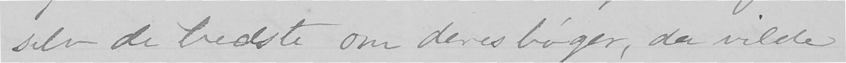selv de bedste om deres bøger, da vilde
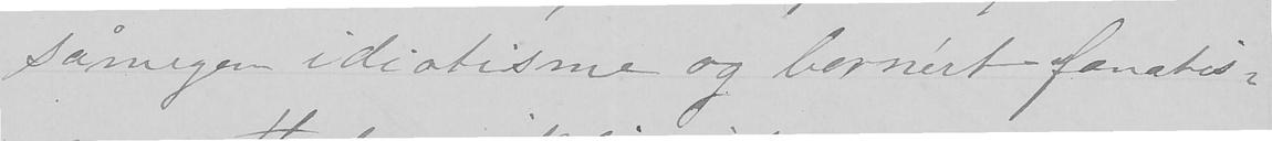såmegen idiotisme og bornért fanatis-
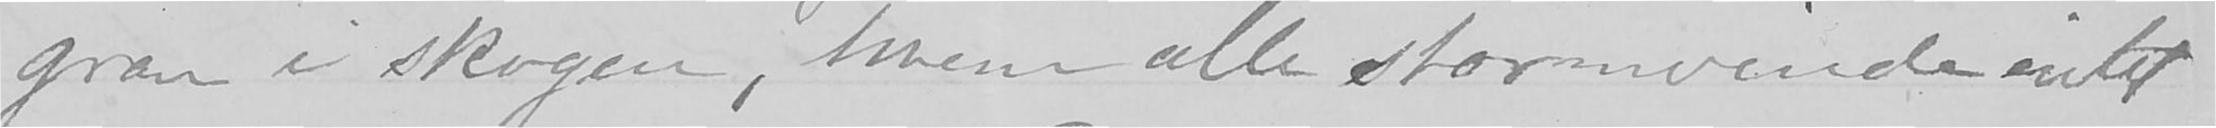gran i skogen, hvem alle stormvinde intet
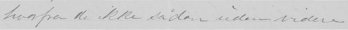hvorfra de ikke sådan uden videre
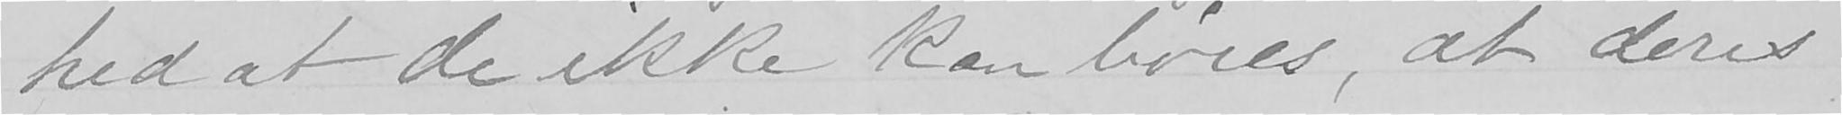hed at de ikke kan bøies, at deres
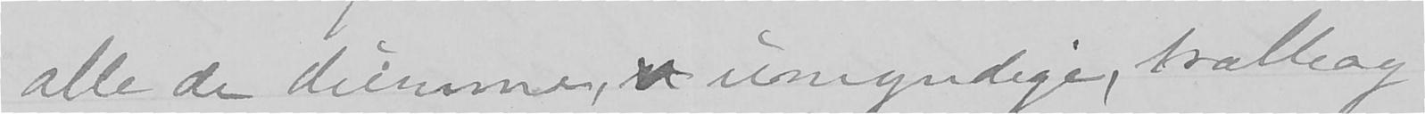alle de dumme, u umyndige, trælleag
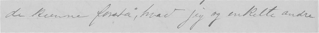de kunne forstå, hvad jeg og enkelte andre
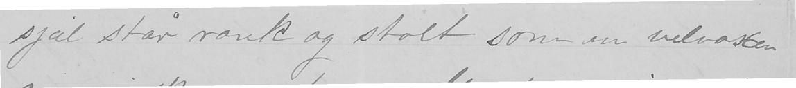sjæl står rank og stolt som en velvoksen
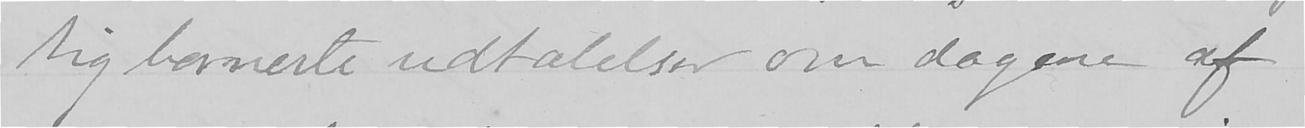tig bornerte udtalelser om dagene af
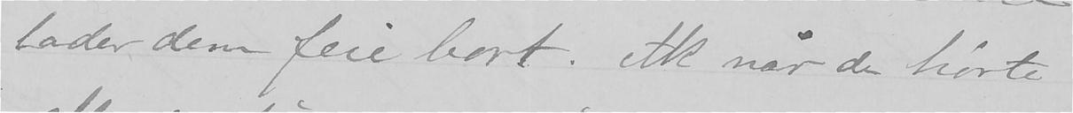lader dem feie bort. Ak når de hørte
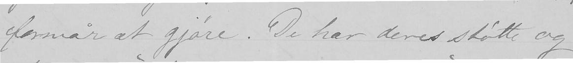formår at gjøre. De har deres støtte og
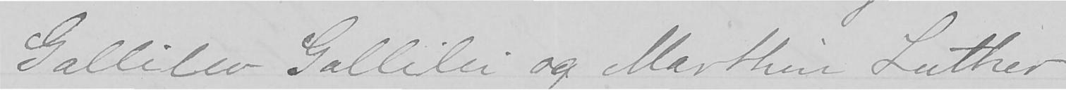Gallileo Gallilei og Marthin Luther
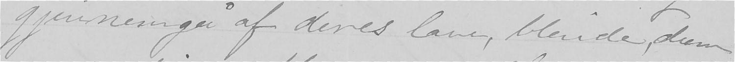gjennemgå af deres lave, blinde dum-
